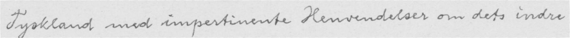Tyskland med impertinente Henvendelser om dets indre
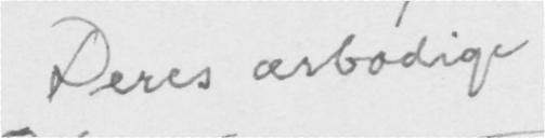Deres ærbødige
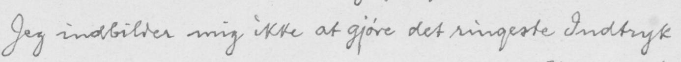Jeg indbilder mig ikke at gjøre det ringeste Indtryk
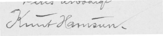Knut Hamsun.
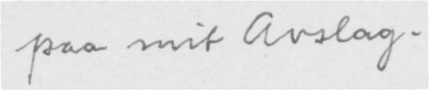paa mit Avslag.
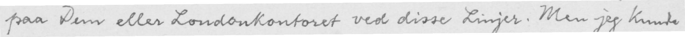paa Dem eller Londonkontoret ved disse Linjer. Men jeg kunde
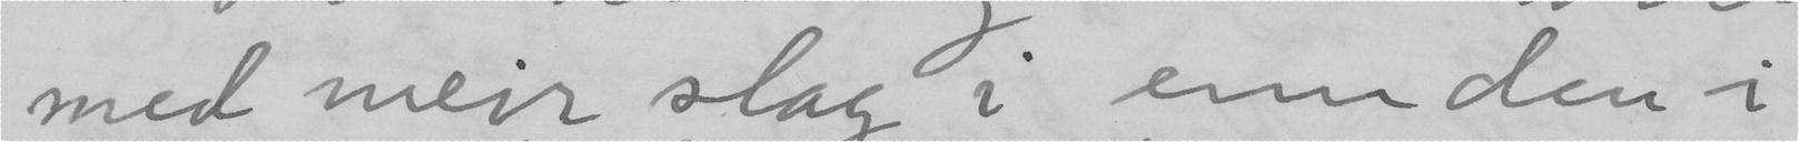med meir slag i enn den i
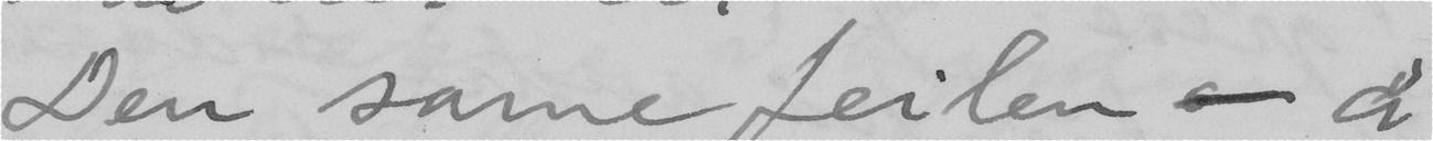Den same feilen – å
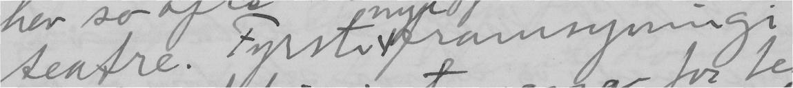teatre. Fyrste nye samsyningi
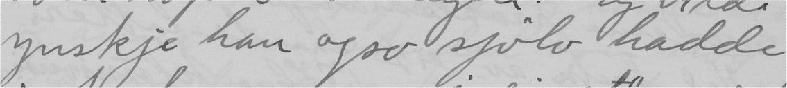ynskje han ogso sjölv hadde
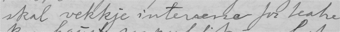skal vekkje interesse for teatre
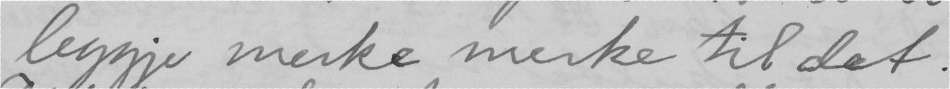leggje merke merke til det.
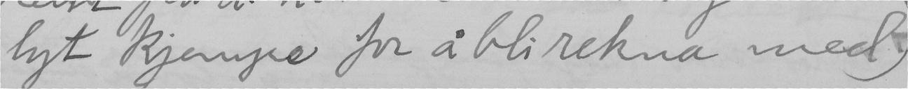lyt kjempe for å bli rekna med.
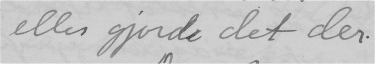elles gjorde det der.
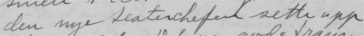den nye teaterchefen sette upp
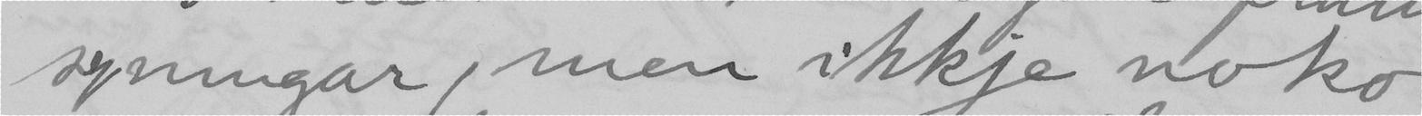syningar, men ikkje noko
